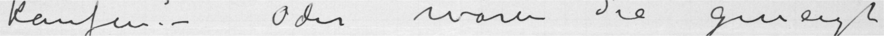kaufen? – Oder wären Sie geneigt
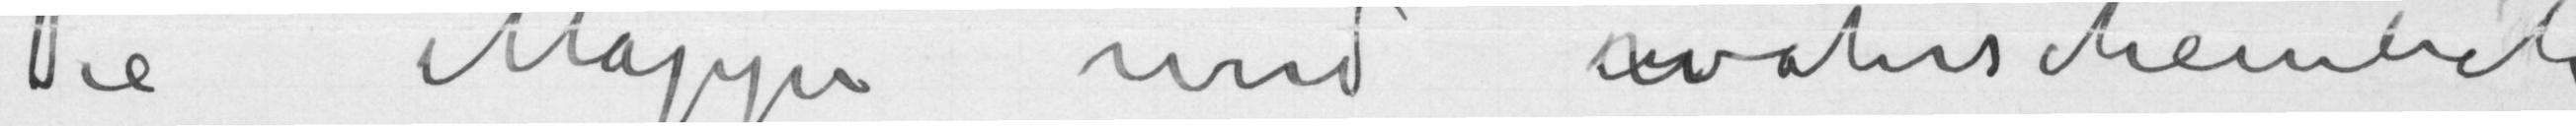Die Mappe und inwahrscheinlich
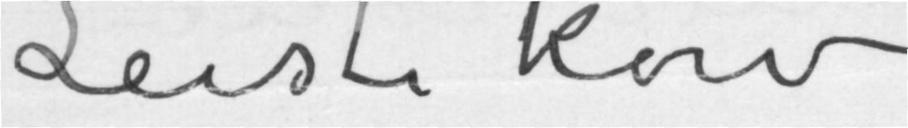Leistikow
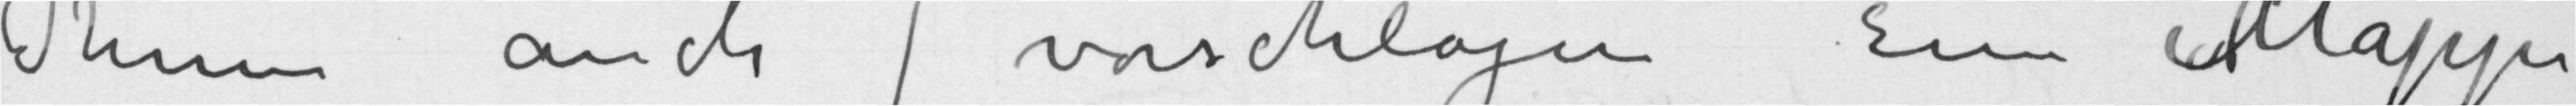Ihnen auch 1 vorschlagen eine Mappe
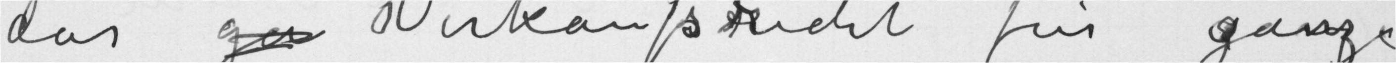das ga Verkaufsrecht für ganz
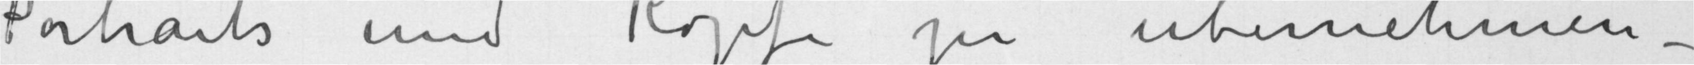Portraits und Kopfe zu ubernehmen –
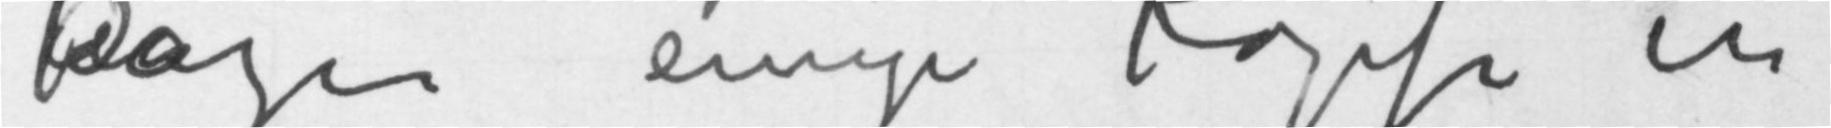einDazu einige Kopfe in
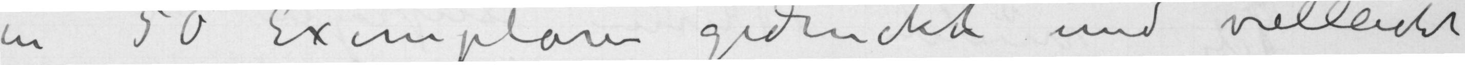in 50 Exemplare gedruckt und vielleicht
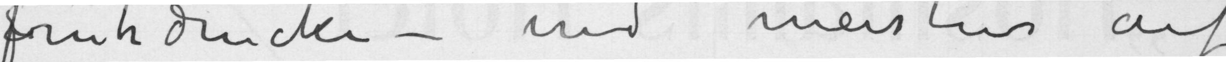Frühdrucke – und meistens auf
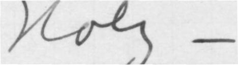Holz
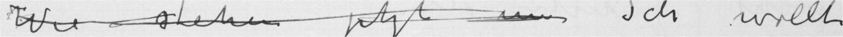Wie stehe jetzt in Ich wollte
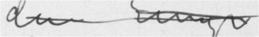den Eingr
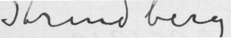Strindberg
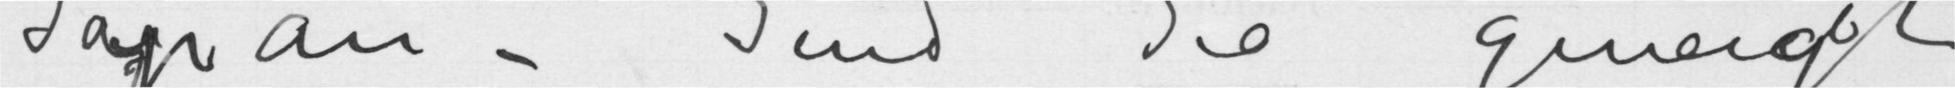Japan – Sind Sie geneidgt
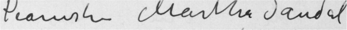Pianistin Martha Sandal
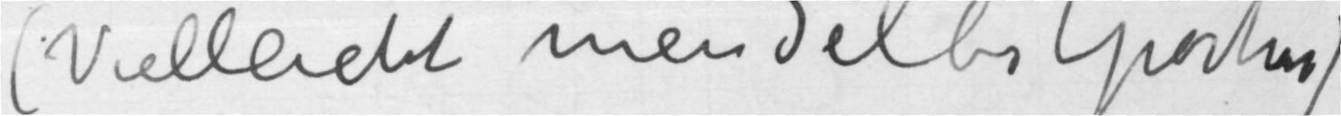(Vielleicht mein Sebstportrait)
